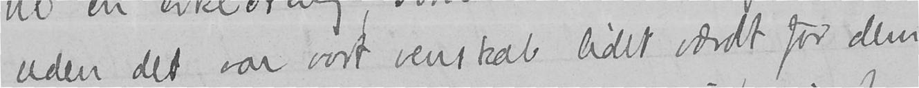uden det var vort venskab lidet værdt for dem,
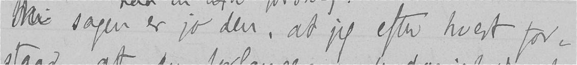Thi sagen er jo den, at jeg efter hvert for-
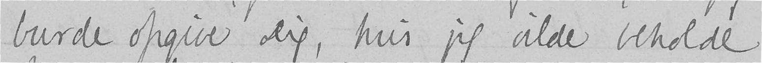burde opgive Dig, hvis jeg vilde beholde
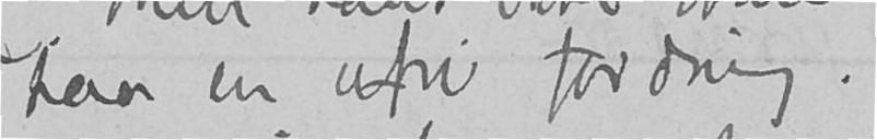paa en ufri tordning.
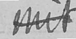mit
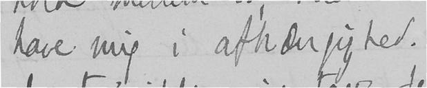have mig i afhængighed.
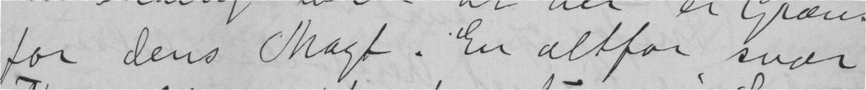for dens Magt. En altfor svær
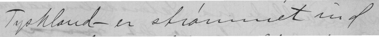Tyskland- er strømmet ind
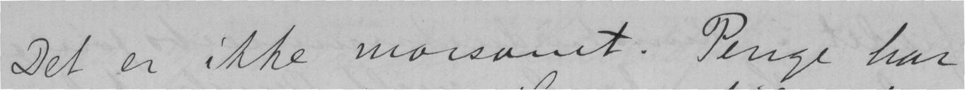Det er ikke morsomt. Penge har
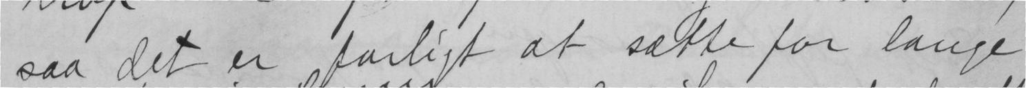saa det er farligt at sætte for lange
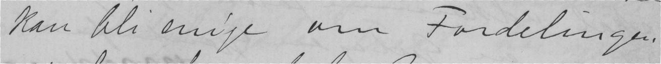kan bli enige om Fordelingen
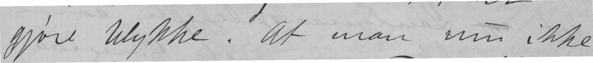gjøre Ulykke. At man nu ikke
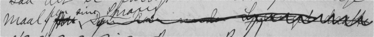maal
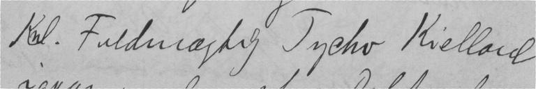Knl. Fuldmægtig Tycho Kielland
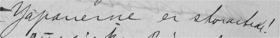Japanerne er storartede!
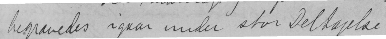begravedes igaar under stor Deltagelse
